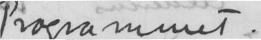Programmet.
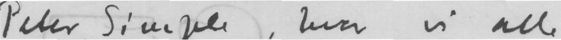Peter Simple, hvor vi alle
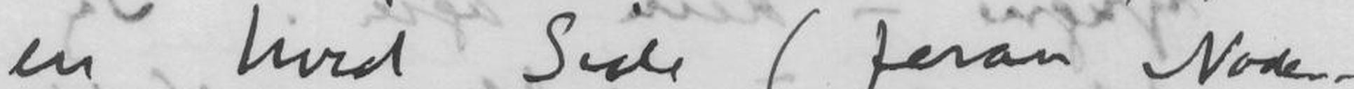en hvid Side (foran Noder-
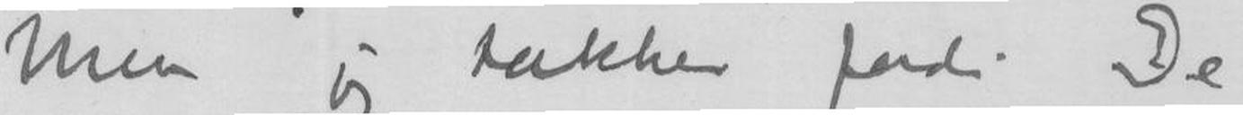Men jeg takker fordi De
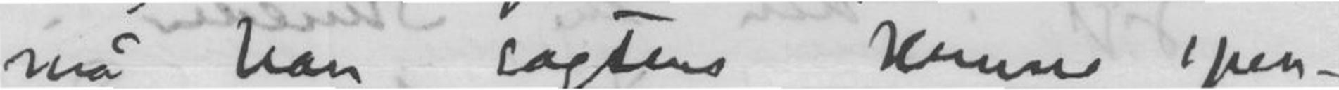må han sagtens kunne spen-
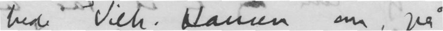bede Vilh. Hansen om, på
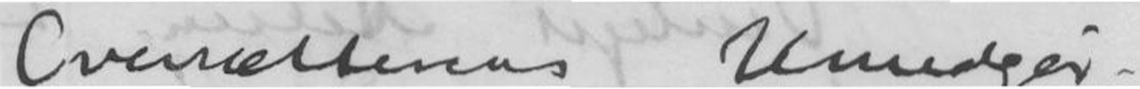Oversætterens Umedgør-
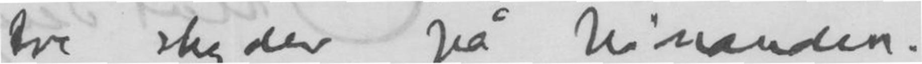tre skylder på hinanden.
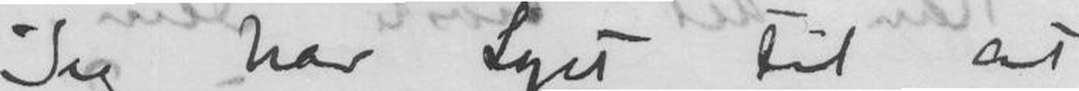Jeg har Lyst til at
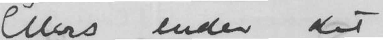Ellers ender det
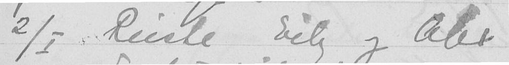2/I Reiste Eileg og Alex
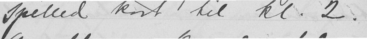spilled kort til kl. 2.
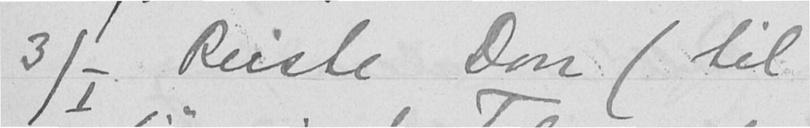3/I Reiste Don (til
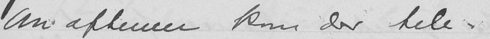Om aftenen kom der tele-
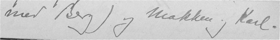med Berg) og Makken og Karl.
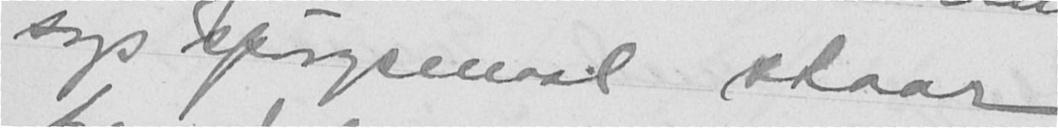sagsspørgsmaal staar
